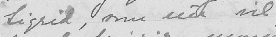Sigrid, som nu vil
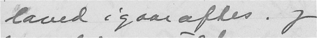laved igaar aftes. og
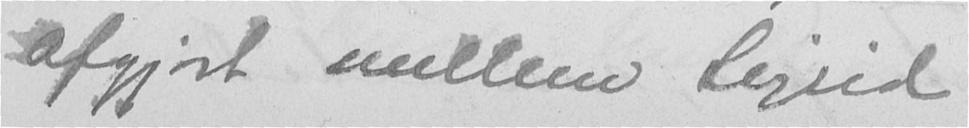afgjort mellem Sigrid
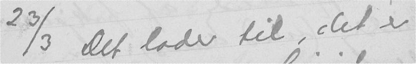23/3 Det lader til, det er
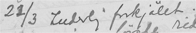22/3 Inderlig forkjølet.
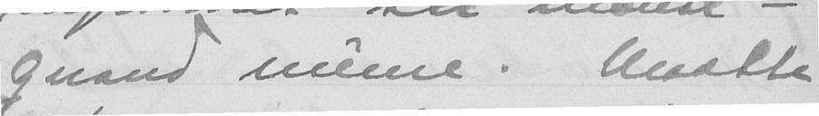quand même. Maatte
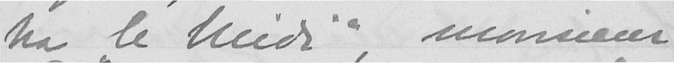ha "le Midi" monsieur
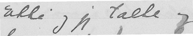Etti og jeg talte og
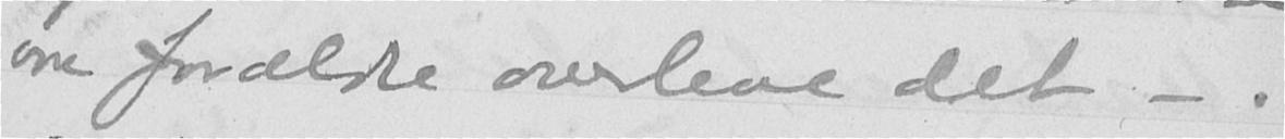vore forældre overleve det -
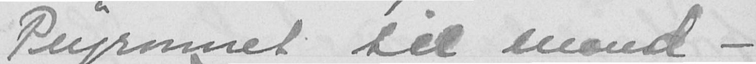Peyronnet til mand -
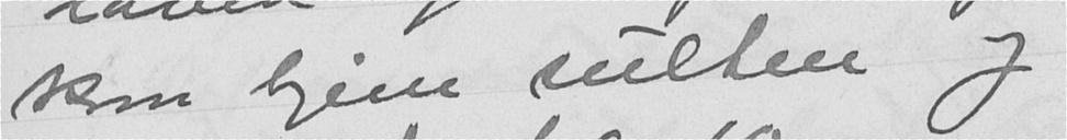kom hjem sulten og
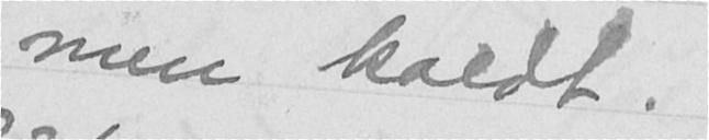men koldt.
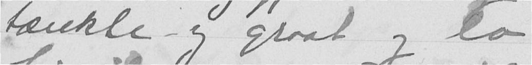tænkte og graat og lo
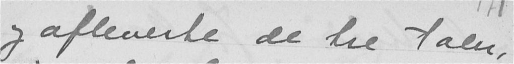og afleverte de tre taler,
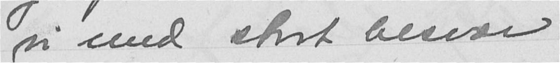vi med stort besvær
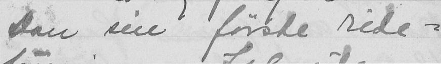Don sin første ride-
